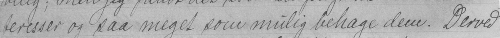teresser og saa meget som mulig behage dem. Derved
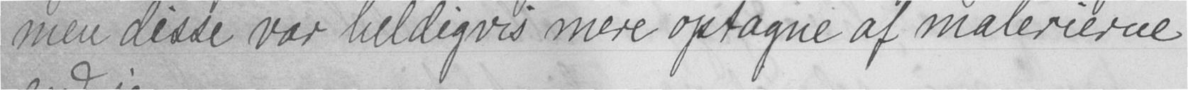men disse var heldigvis mere optagne af malerierne
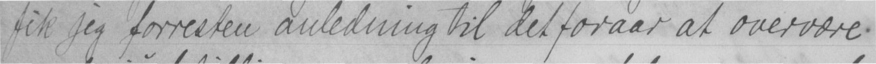fik jeg forresten anledning til det foraar at overvære
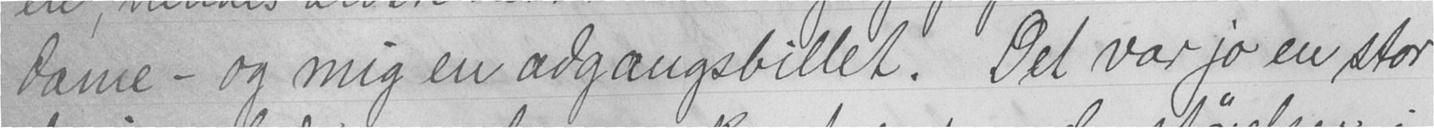dame - og mig en adgangsbillet. Det var jo en stor
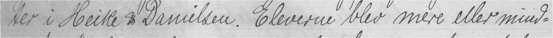ter i Heike & Danielsen. Eleverne blev mere eller mind-
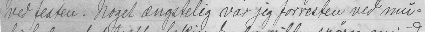ved festen. Noget ængstelig var jeg forresten ved mu-
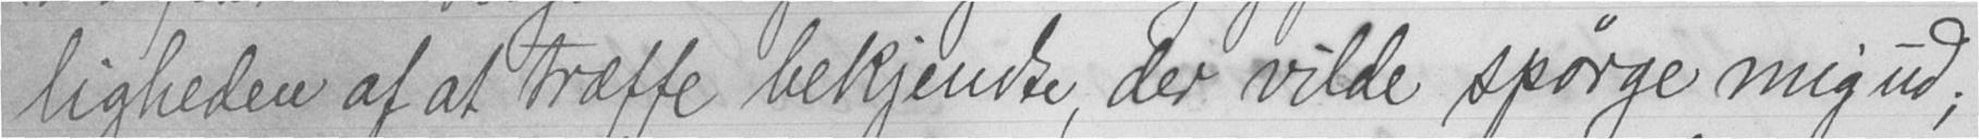ligheden af at træffe bekjendte, der vilde spørge mig ud;
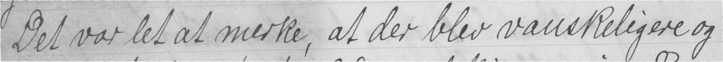Det var let at merke, at der blev vanskeligere og
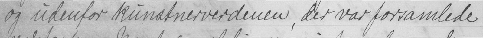og udenfor kunstnerverdenen, der var forsamlede
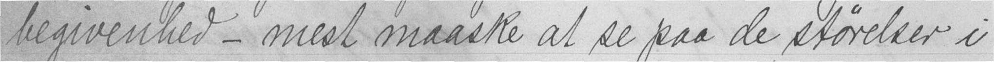begivenhed - mest maaske at se paa de størelser i
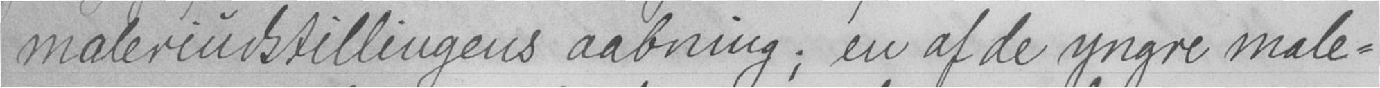maleriudstillingens aabning; en af de yngre male-
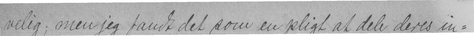velig; men jeg fandt det som en pligt at dele deres in-
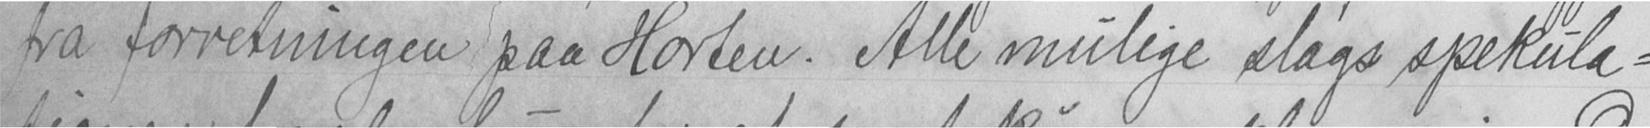fra forretningen paa Horten. Alle mulige slags spekula-
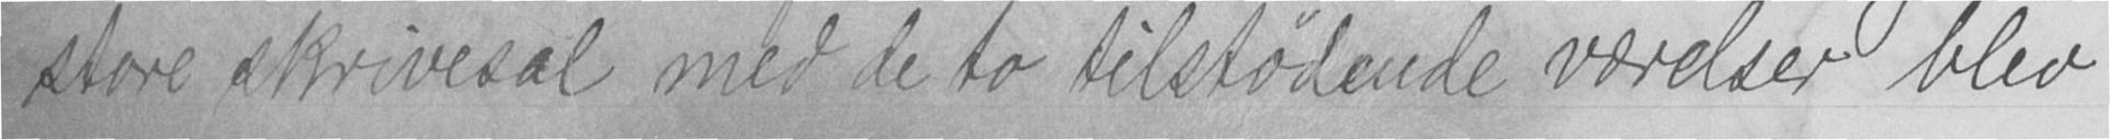store skrivesal med de to tilstødende værelser blev
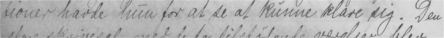tioner havde hun for at se at kunne klare sig. Den
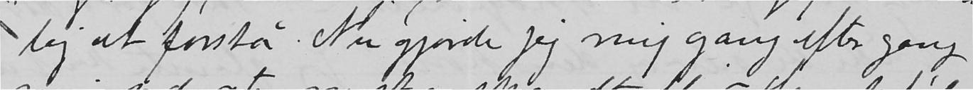lig at forstå. Nu gjorde jeg mig gang efter gang
